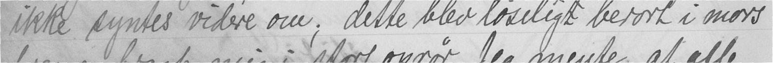ikke syntes videre om; dette blev løseligt berørt i mors
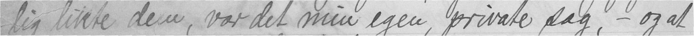lig likte dem, var det min egen, private sag - og at
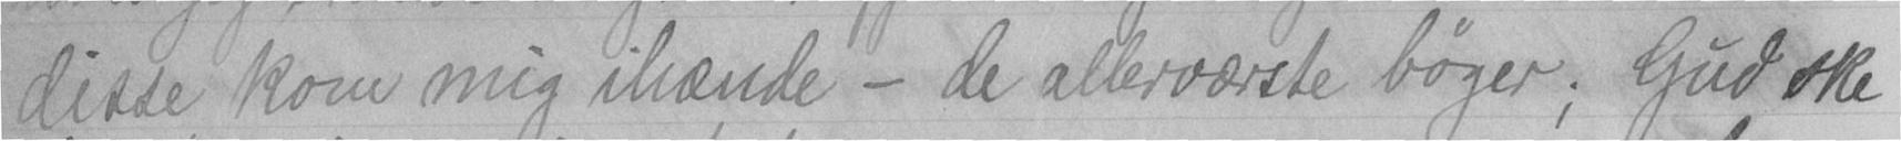disse kom mig ihænde - de allerværste bøger; Gud ske
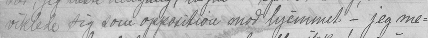viklede sig som opposition mod hjemmet - jeg me-
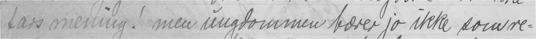fars mening! men ungdommen bærer jo ikke som re-
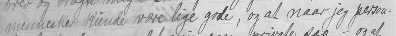mennesker kunde være lige gode, og at naar jeg person-
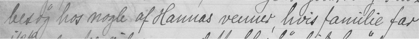besøg hos nogle af Hannas venner, hvis familie far
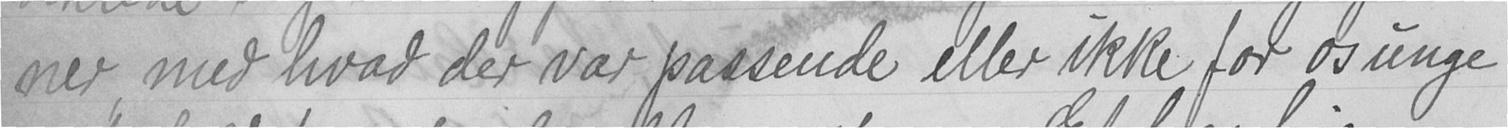ner, med hvad der var passende eller ikke for os unge
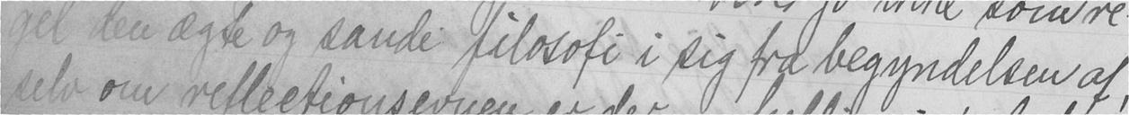gel den ægte og sande filosofi i sig fra begyndelsen af,
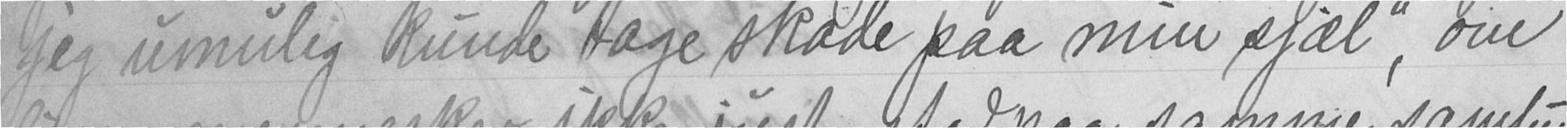jeg umulig kunde "tage skade paa min sjæl", om
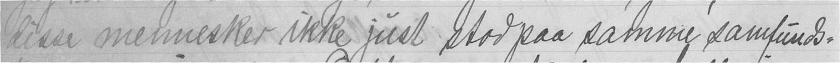disse mennesker ikke just stod paa samme samfunds-
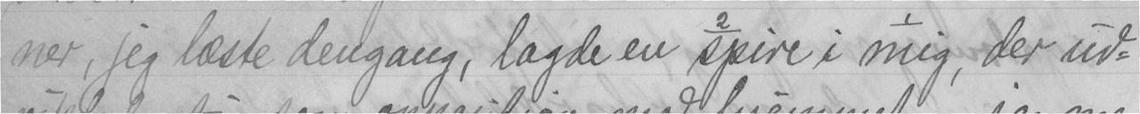ner, jeg læste dengang, lagde en spire i mig, der ud-
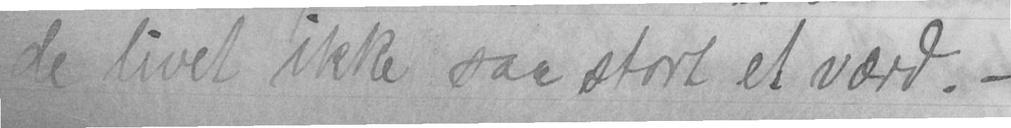de livet ikke saa stort et værd. -
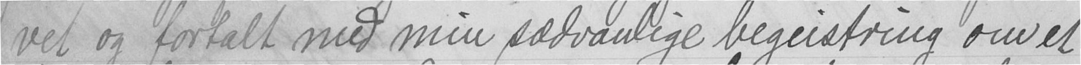vet og fortalt med min sædvenlige begeistring om et
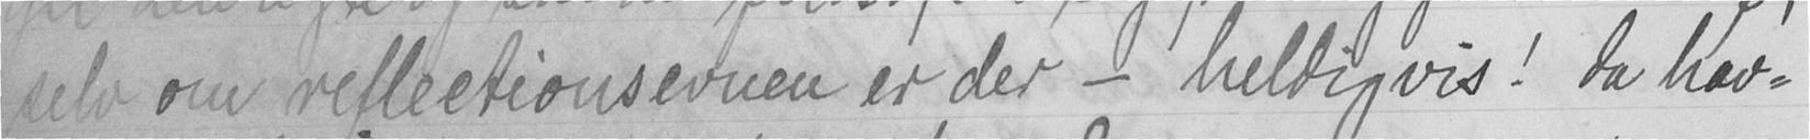selv om reflectionsevnen er der - heldigvis! da hav-
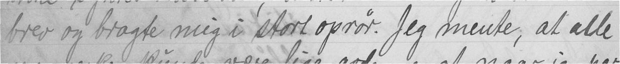brev og bragte mig i stort oprør. Jeg mente, at alle
